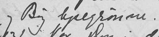og Bøg lysegrønne.
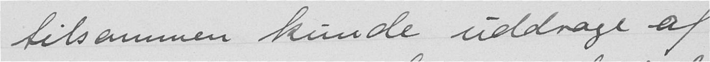tilsammen kunde uddrage af
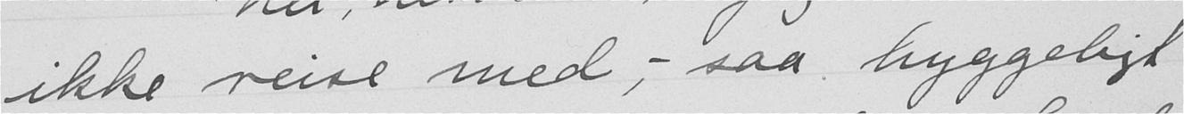ikke reise med, - saa hyggeligt
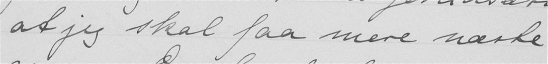at jeg skal faa mere næste
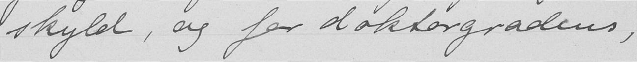skyld, og for doktorgradens,
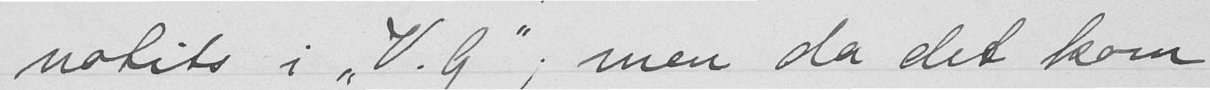notits i "V.G"; men da det kom
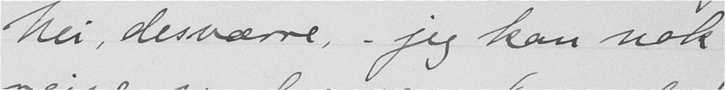Nei, desværre, - jeg kan nok
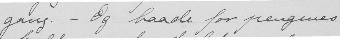gang. - Og baade for pengenes
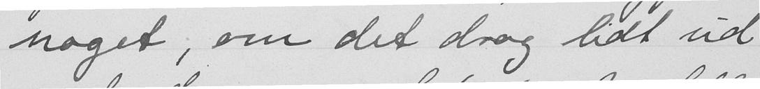noget, om det drog lidt ud
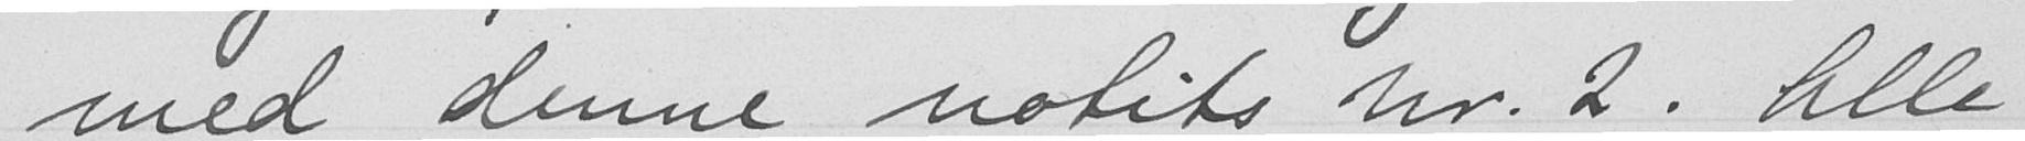med denne notits nr. 2. Alle
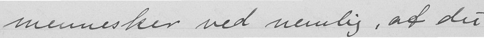mennesker ved nemlig, at du
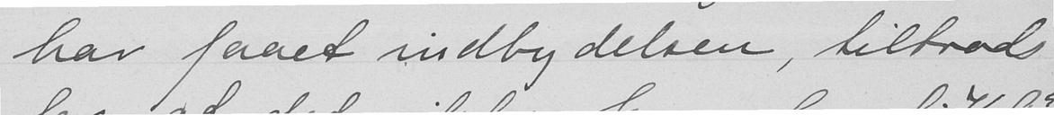har faaet indbydelsen, tiltrods
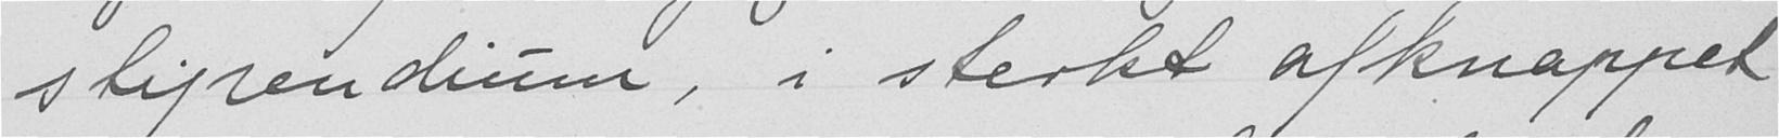stipendium, i sterkt afknappet
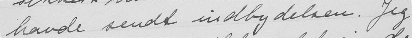havde sendt indbydelsen. Jeg
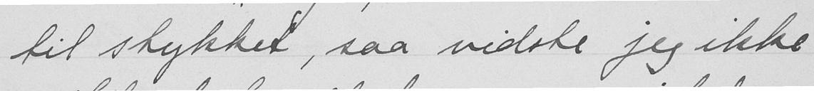til stykket, saa vidste jeg ikke
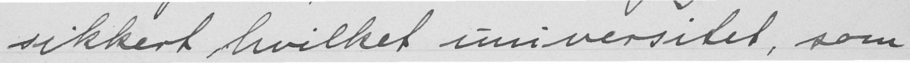sikkert hvilket universitet, som
